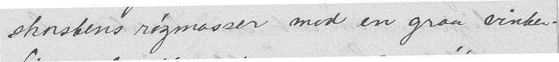skorstens røgmasser med en graa vinter-
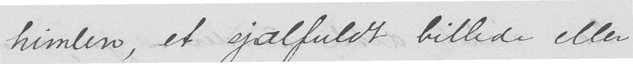himlen, et sjælfuldt billede eller
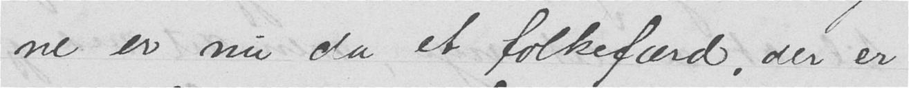ne er nu da et folkefærd, der er
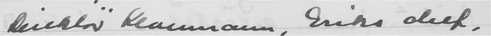Direktør Klanmann, Eriks chef,
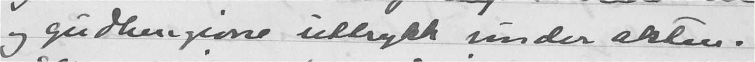og gudhengivne uttrykk under akten.
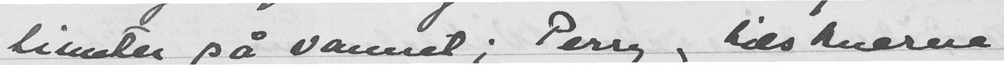timeter på vannet; Perry og Nils knern
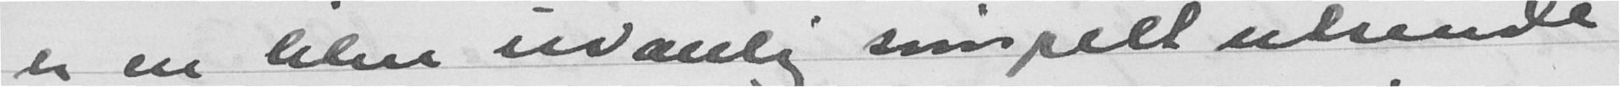er en lilen uvanlig simpelt utseende
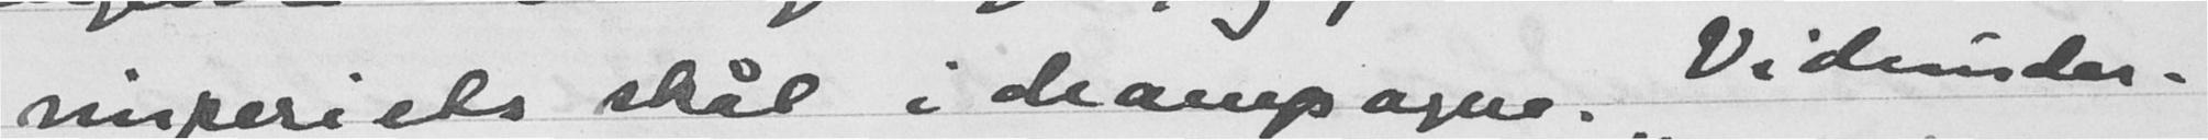imperiets skål i champagne. Vidunder-
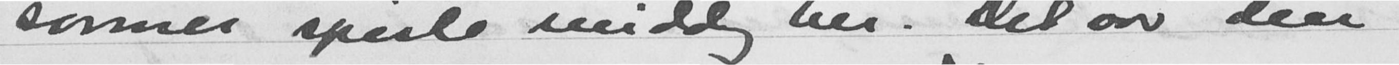sønner spiste middag her. Det var den
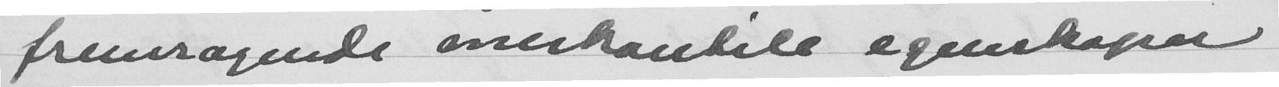fremragende veskantele egenskaper
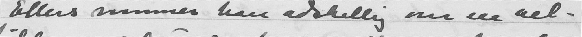Ellers minner han adskillig om en hel-
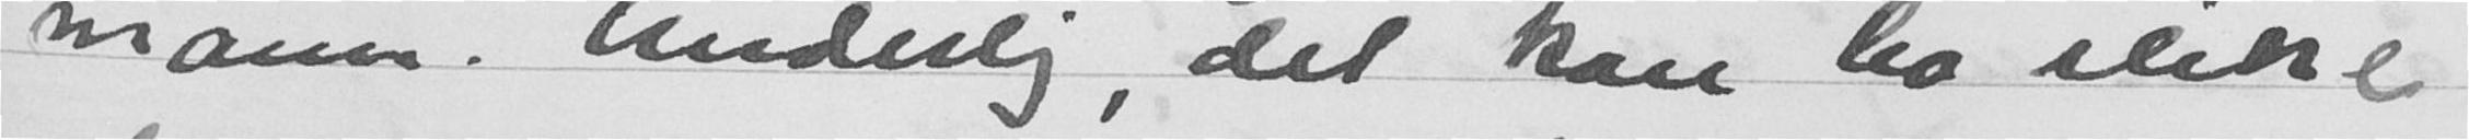mann. Underlig det kan ha slike
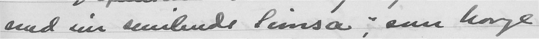med sin smilende Timsa; som Norge
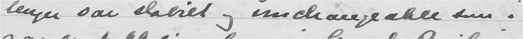lenger var stabilt og unchangeable som i
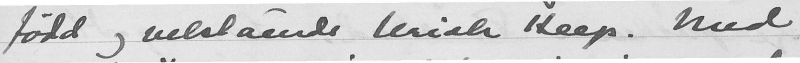fødd og velstående Uriah Heep. Med
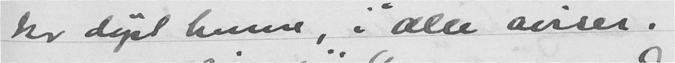har døpt henne, i alle aviser.
